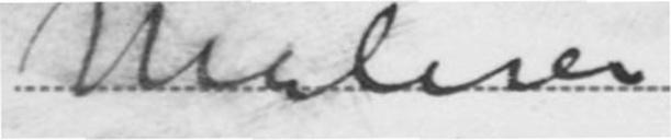Maleren
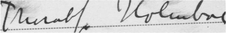Thorolf Holmboe
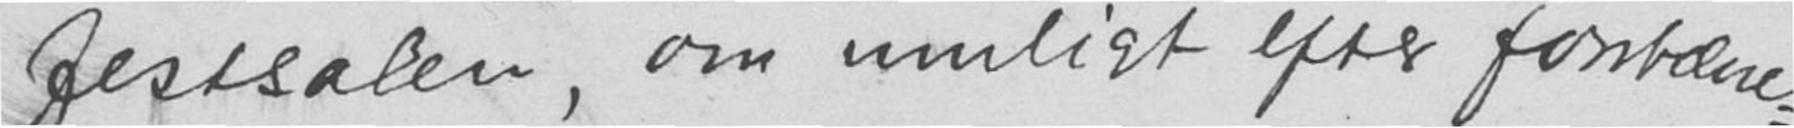festsalen, om muligt efter fontæne-
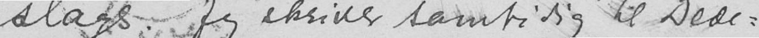slags. Jeg skriver samtidig til Dede-
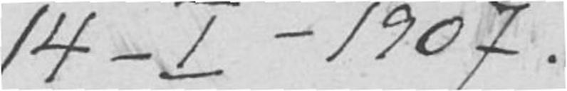14-1-1907.
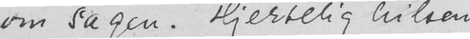om sagen. Hjertelig hilsen
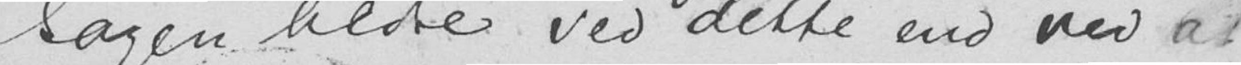sagen bedre ved dette end ved at
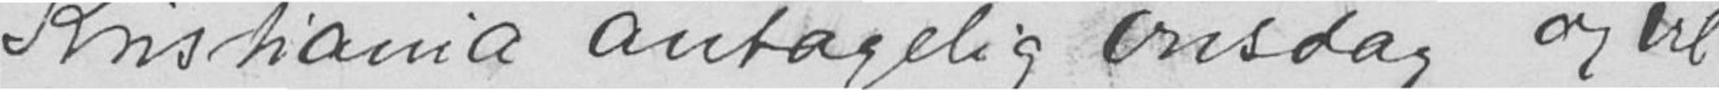Kristiania antagelig onsdag og vil
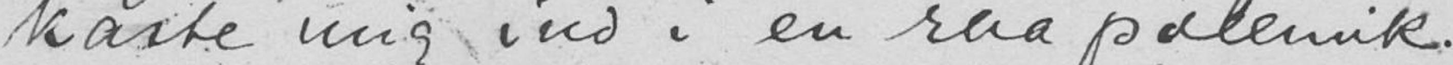kaste mig ind i en raa polemik.
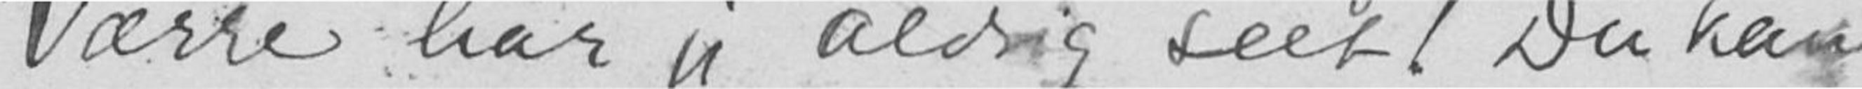Værre har jeg aldrig seet! Du kan
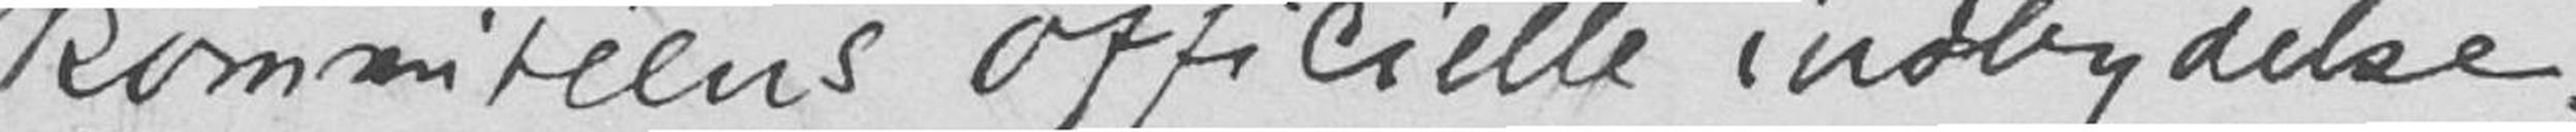kommitéens officielle indbydelse.
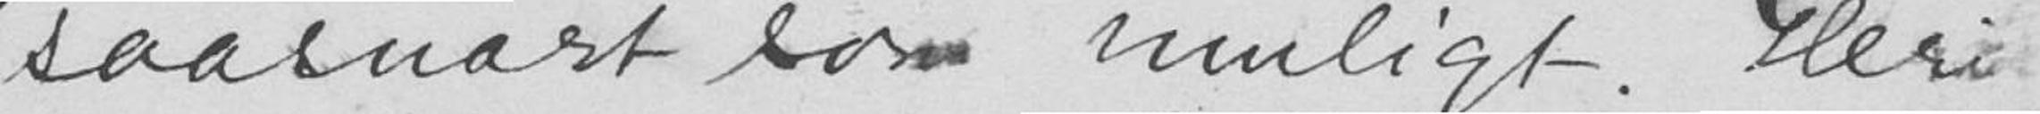saasnart som muligt. Heri
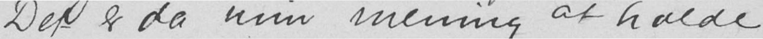Det er da min mening at holde
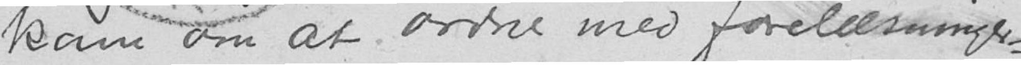kam om at ordne med forelæsninger-
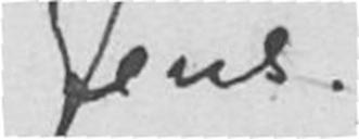Jens.
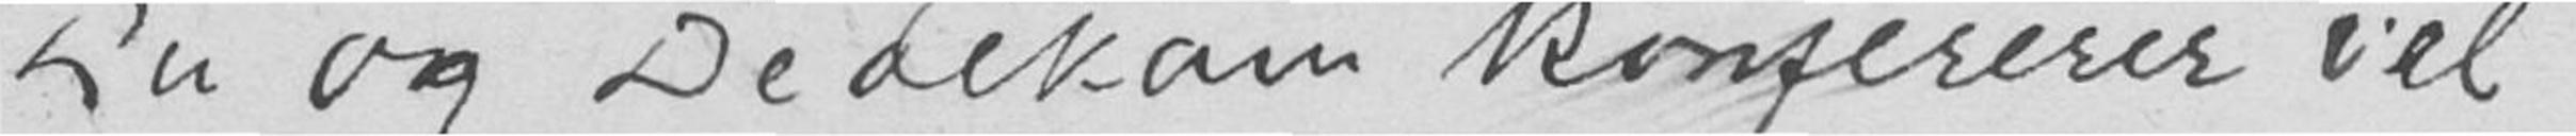Du og Dedekam konfererer vel
